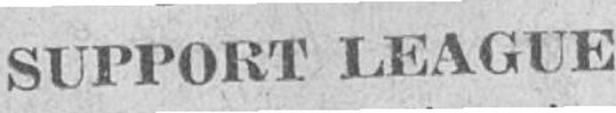SUPPORT LEAGUE
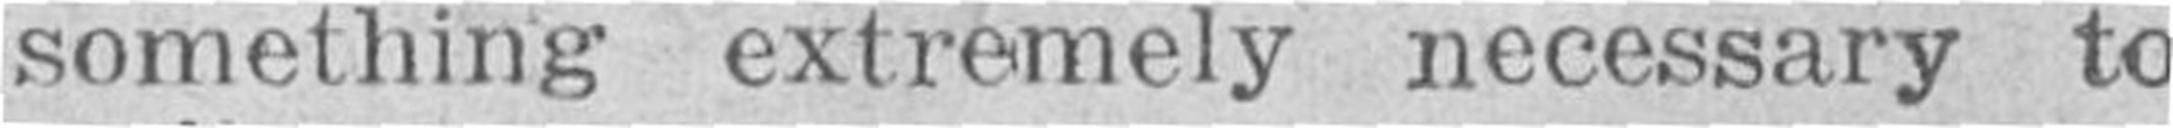something extremely necessary to
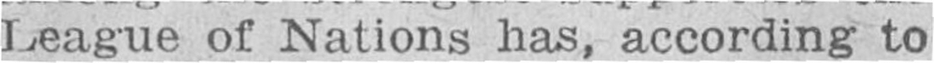League of Nations has, according to
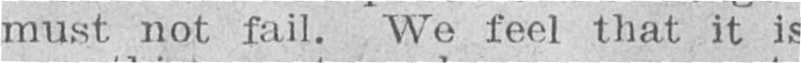must not fail. We feel that it is
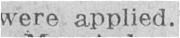were applied."
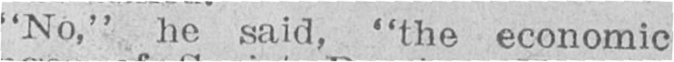"No," he said, "the economic
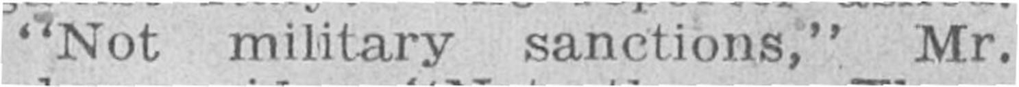"Not military sanctions," Mr.
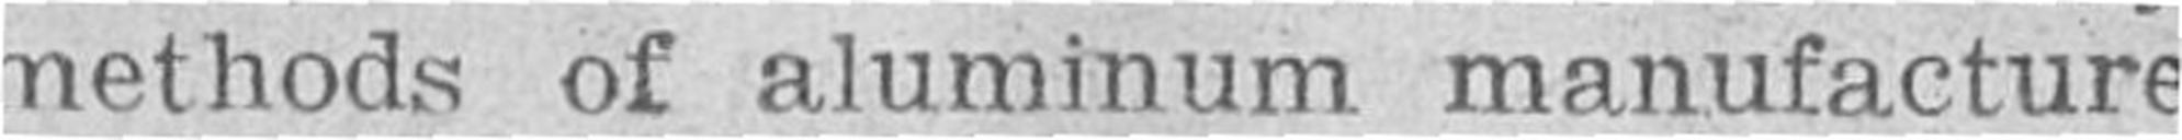methods of aluminum manufacture
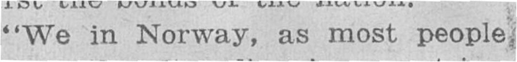"We in Norway, as most people
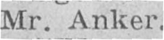Mr. Anker,
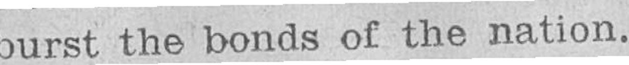nurst the bonds of the nation.
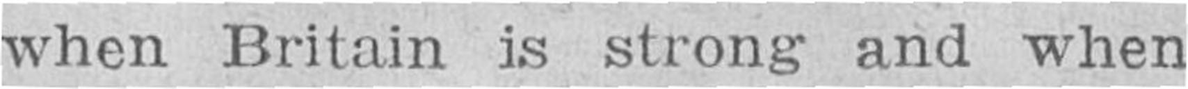when Britain is strong and when
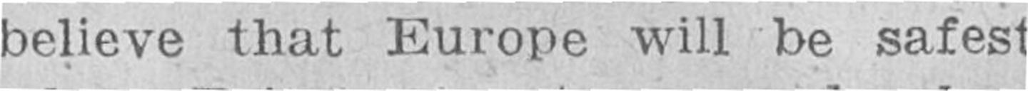believe that Europe will be safest
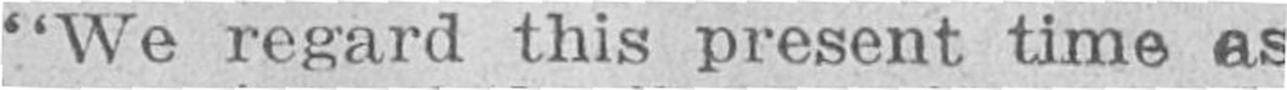"We regard this present time as
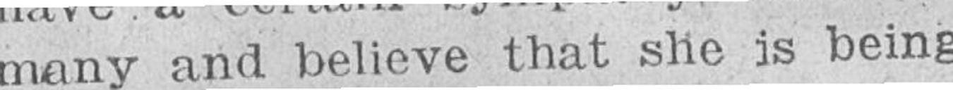many and believe that she is being
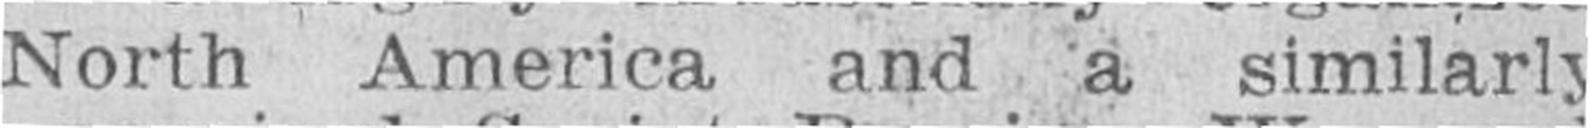North America and a similarly
